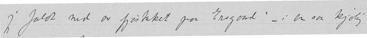jeg faldt ned av fjøstaket paa Ersgaard" —; i en kjølig
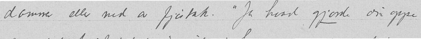dammer eller ned av fjøstak. "Ja hvad gjorde du oppe
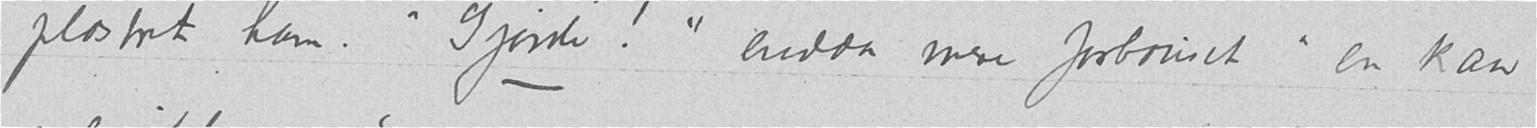plastret ham. "Gjorde!" endda mere forbauset "en kan
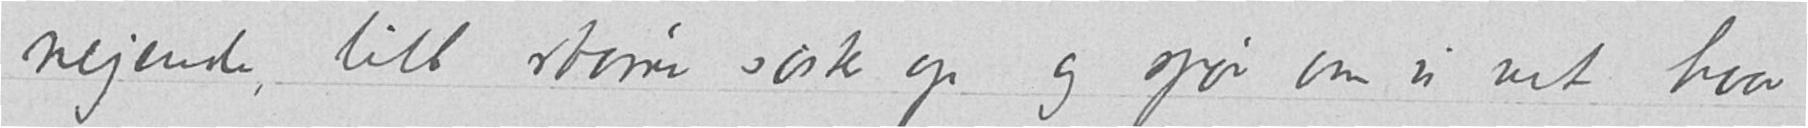nejende, lit større søster op og spør om vi vet hvor
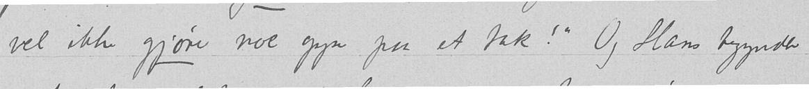vel ikke gjøre noe oppe paa et tak!" Og Hans begynder
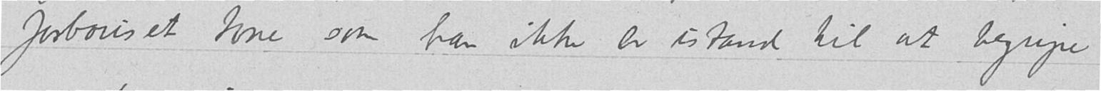forbauset tone som han ikke er istand til at begripe,
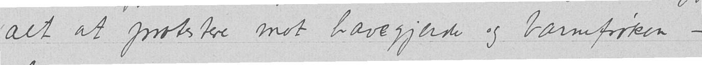alt at protestere mot havegjerder og barnefrøken —
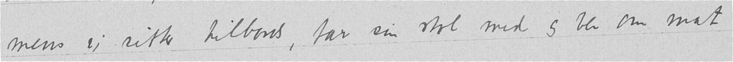mens vi sitter tilbords, tar sin stol med og ber om mat
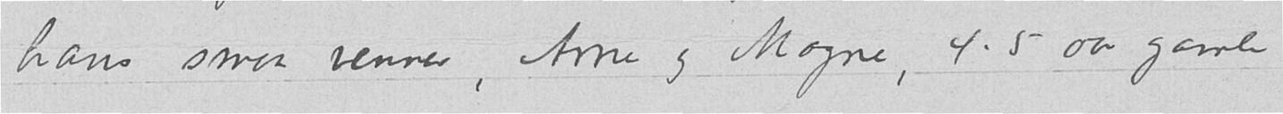hans smaa venner, Arne og Magne, 4-5 aar gamle
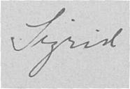Sigrid
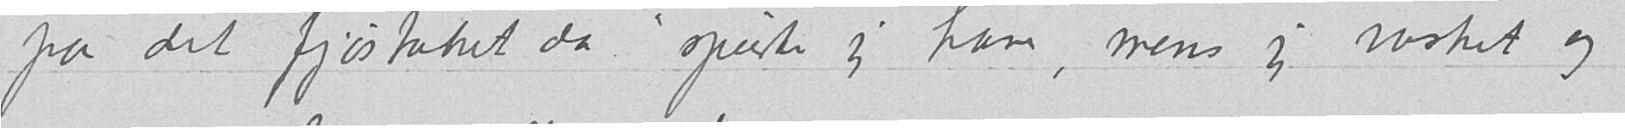på det fjøstaket da" spurte jeg ham, mens jeg vasket og
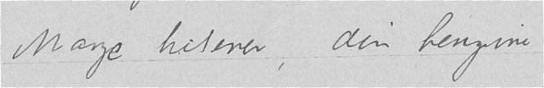Mange hilsener, din hengivne
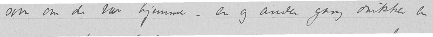som om de var hjemme — en og anden gang dukker en
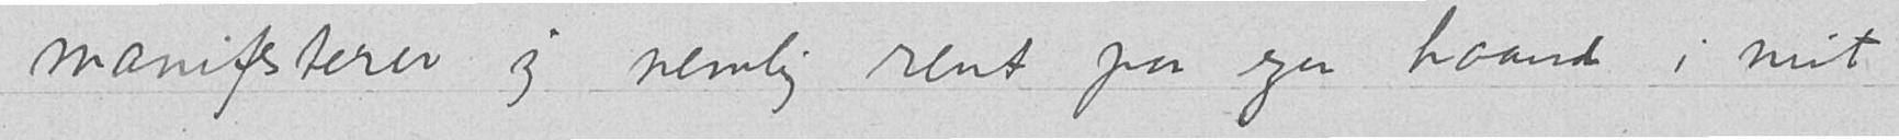manifesterer sig nemlig rent paa egen haand i mit
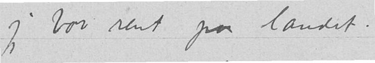jeg bor rent paa landet.
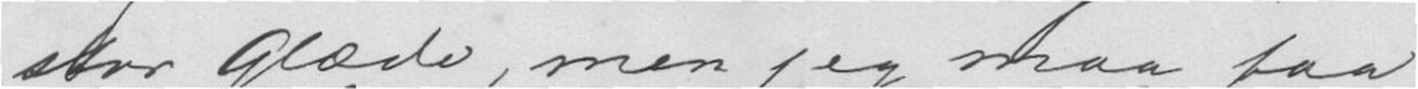stor Glæde, men jeg maa faa
